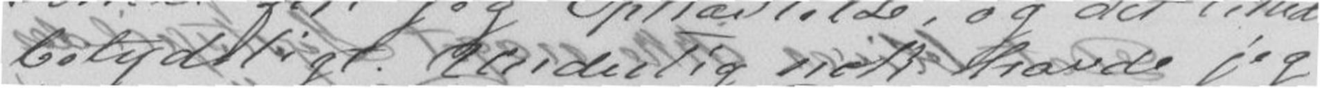betydeligt. Underlig nok havde jeg
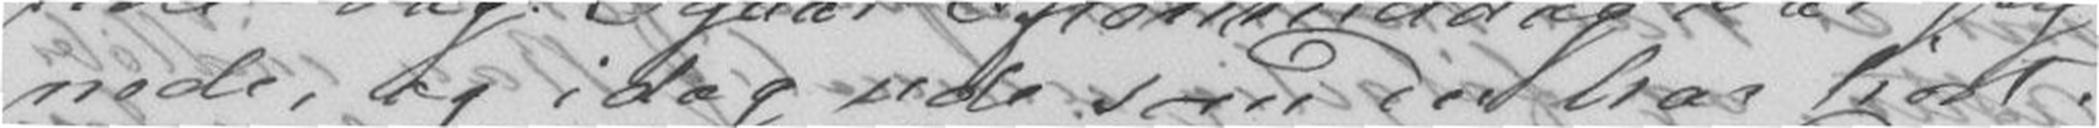nede, og idag ude som Du har hørt.
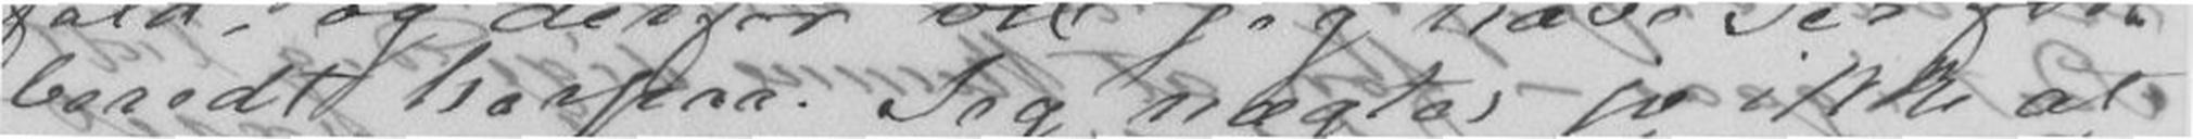beredt herpaa. Jeg nægter jo ikke at
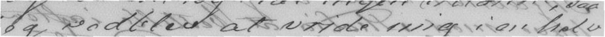jeg vedblev at vride mig i en halv
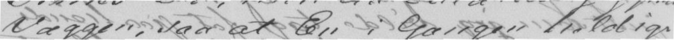Væggen, saa at En i Gangen heldig-
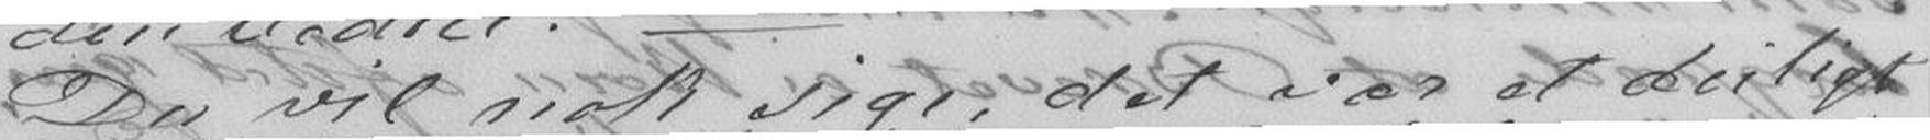Du vil nok sige, det var et deiligt
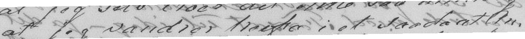at jeg vandrer herfra i et saadant An-
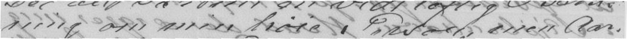ning om min høie Person, men Aar-
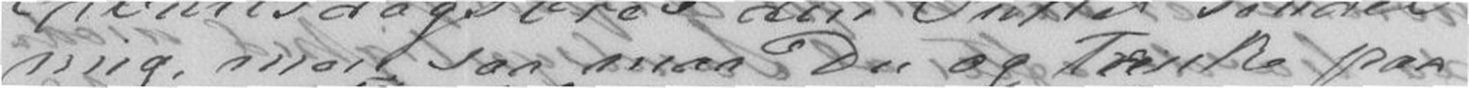mig, men saa maa Du og tænke paa
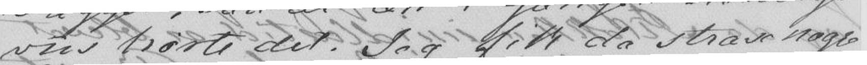viis hørte det. Jeg fik da strax nogle
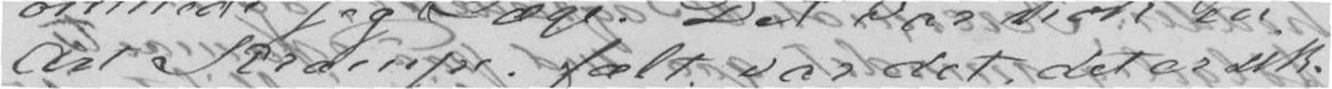Art Krampe, fælt var det, det er sik-
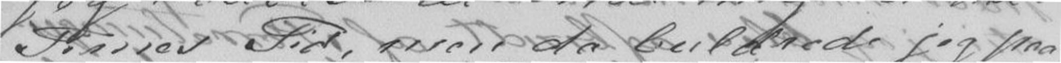Times Tid, men da buldrede jeg paa
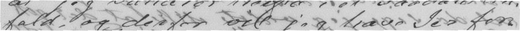fald, og derfor vil jeg have Jer for-
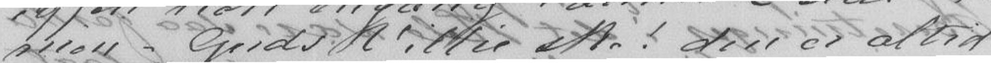men Guds Villie ske! den er altid
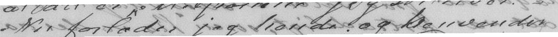Nu forlader jeg hende og henvender
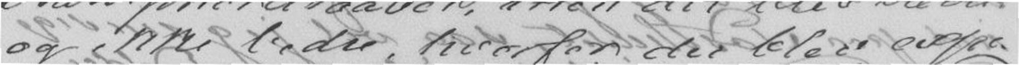og ikke bedre, hvorfor der blev expe-
